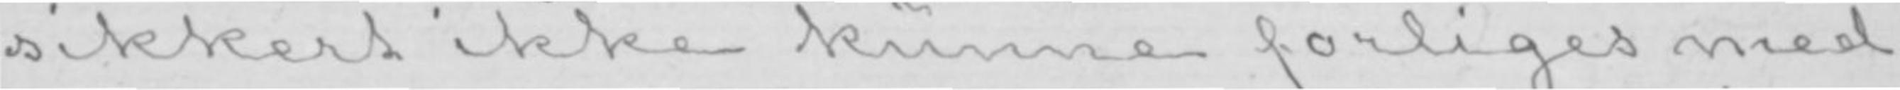sikkert ikke kunne forliges med
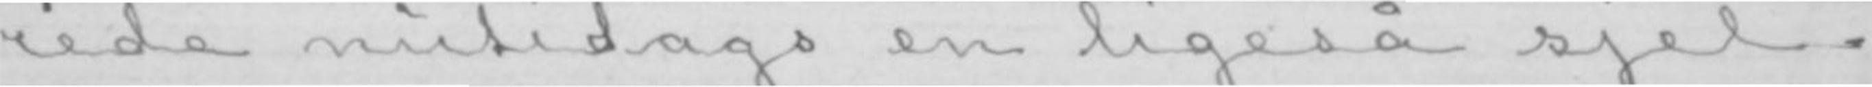rede nutildags en ligeså sjel-
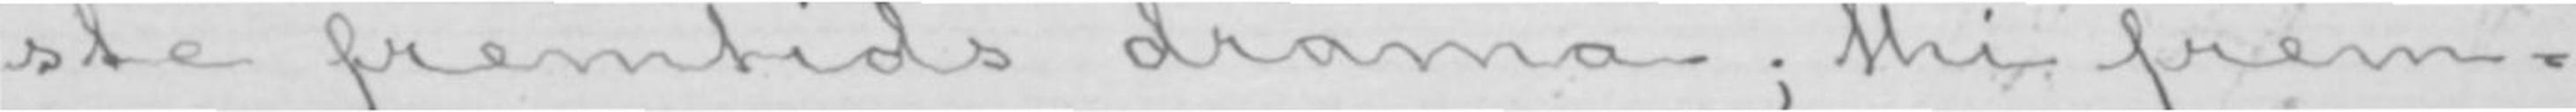ste fremtids drama; thi frem-
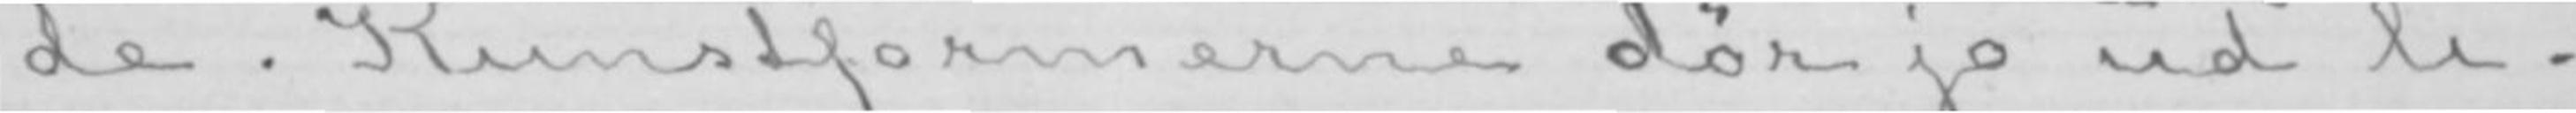de. Kunstformerne dør jo ud li-
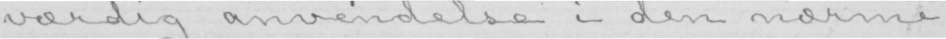værdig anvendelse i den nærme-
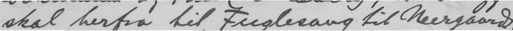skal herfra til Fuglesang til Neergaards,
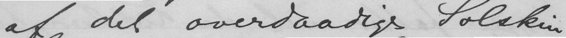af det overdaadige Solskin
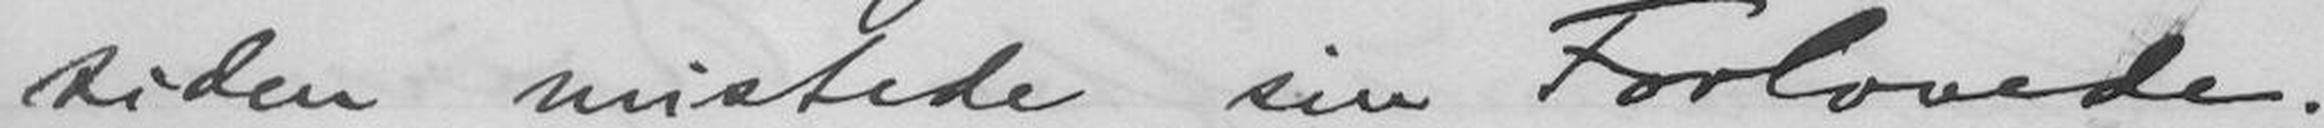siden mistede sin Forlovede.
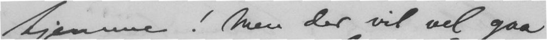hjemme! Men der vil vel gaa
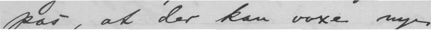pas, at der kan voxe nye
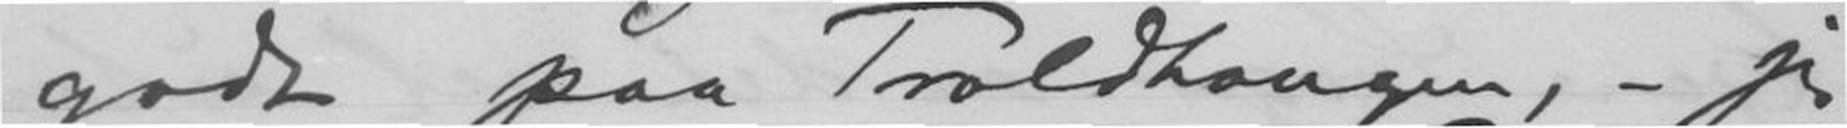godt paa Troldhaugen, - jeg
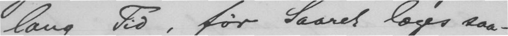lang Tid, før Saaret læges saa-
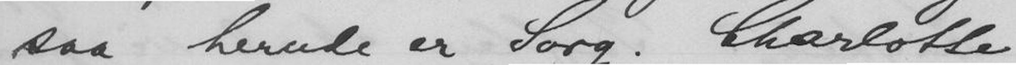saa herude er Sorg. Charlotte
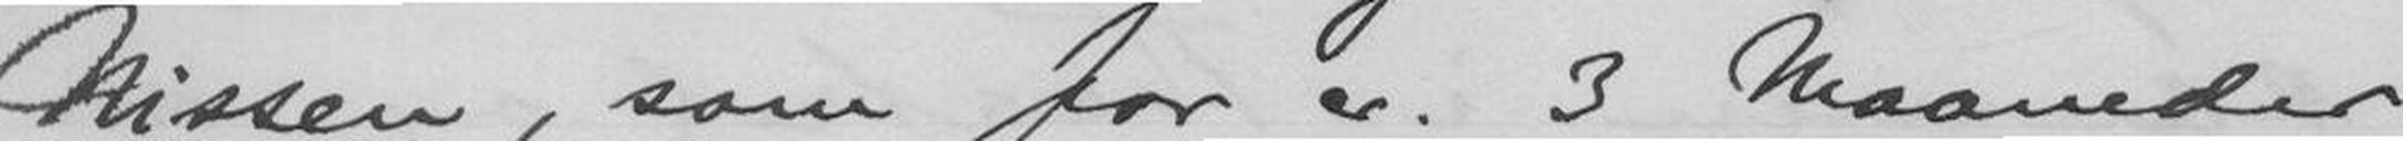Nissen, som for ca. 3 Maaneder
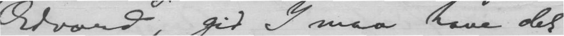Edvard, gid I maa have det
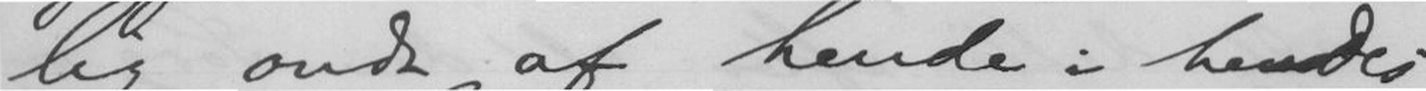lig ondt af hende i hendes
